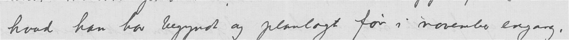hvad han har begyndt og planlagt før i november engang,
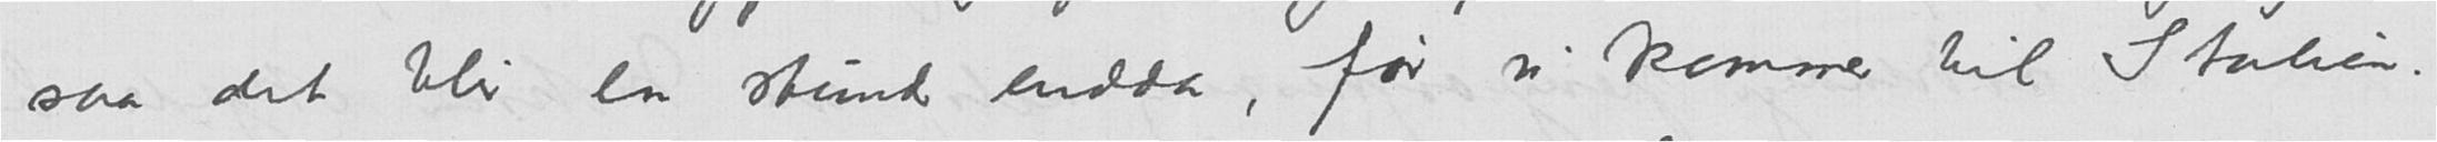saa det blir en stund endda, før vi kommer til Italien.
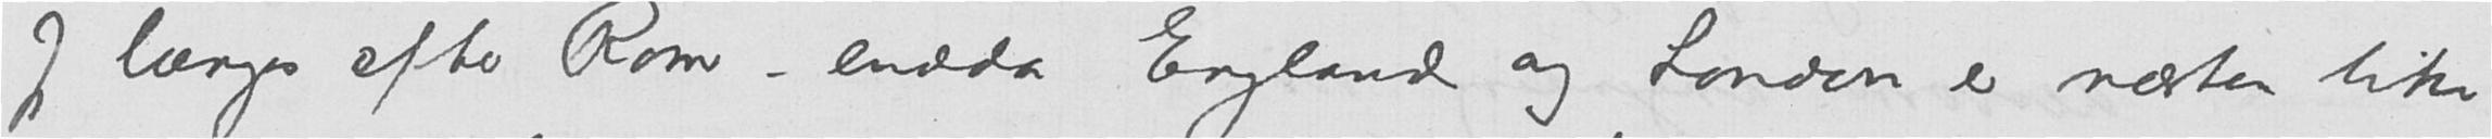Jeg længes efter Rom – endda England og London er næsten like
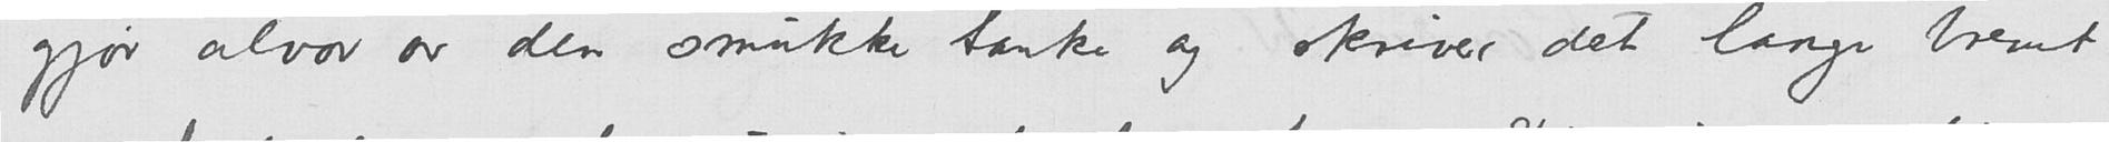gjør alvor av den smukke tanke og skriver det lange brevet
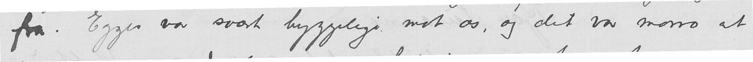fra. Egges var svært hyggelige mot os, og det var morro at
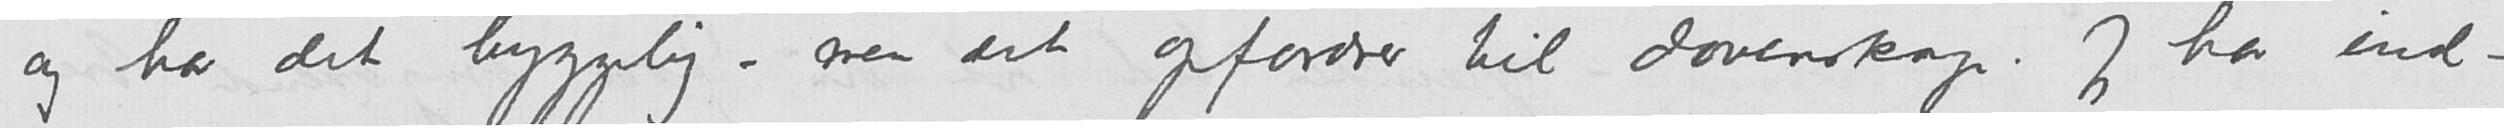og har det hyggelig – men det opfordrer til dovenskap. Jeg har ind-
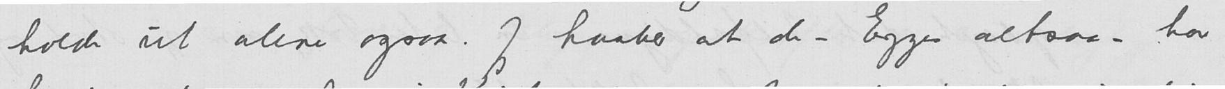holde ut alene ogsaa. Jeg haaber at de – Egges altsaa – har
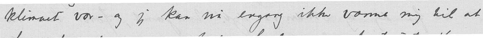klimaet var - og jeg kan nu engang ikke vænne mig til at
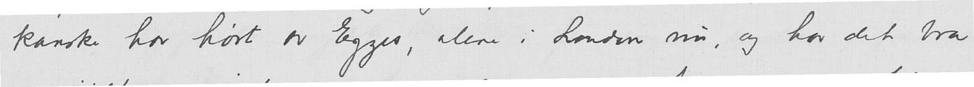kanske har hørt av Egges, alene i London nu, og har det bra
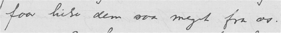faar hilse dem saa meget fra os.
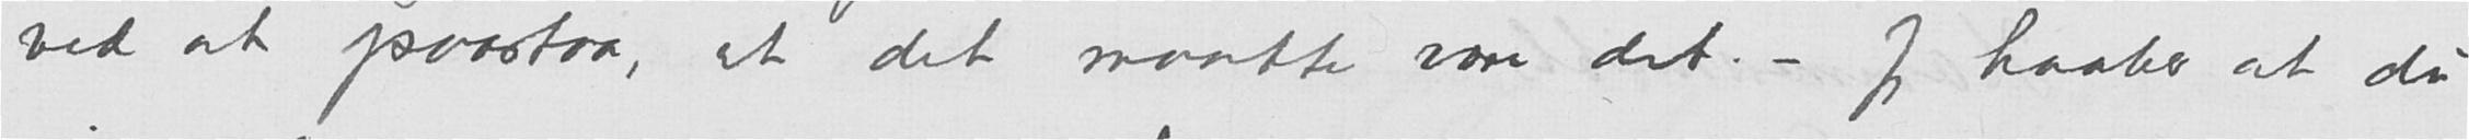ved at paastaa, at det maatte være det. – Jeg haaber at du
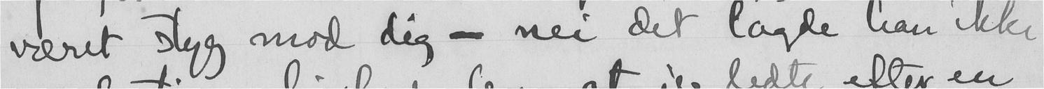været styg mod dig, - nei det lagde han ikke
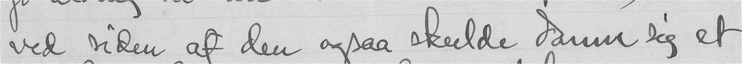ved siden af dem ogsaa skulde danne sig et
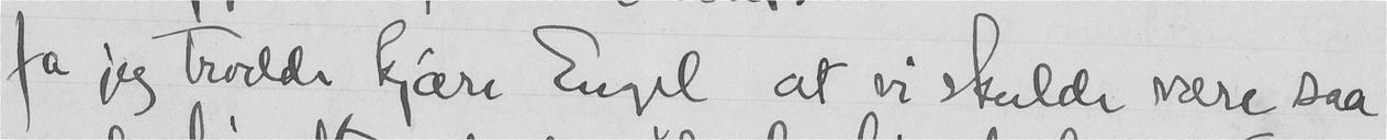Ja jeg trodde kjære Engel at vi skulde være saa
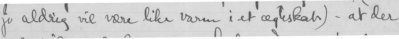jo aldrig vil være like varm i et ægteskab), - at der
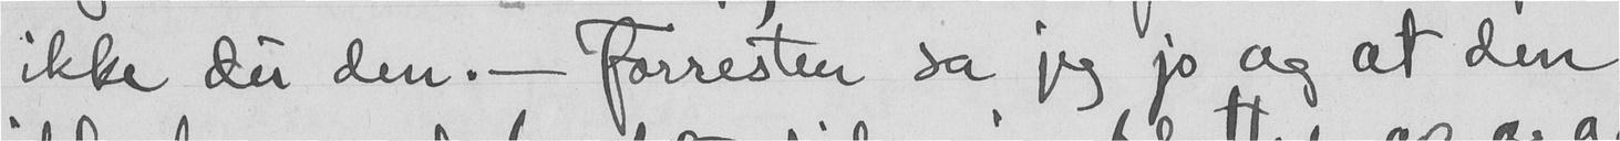ikke du den. - Forresten sa jeg jo og at den
